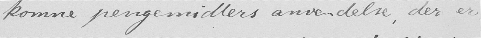komne pengemidlers anvendelse, der er
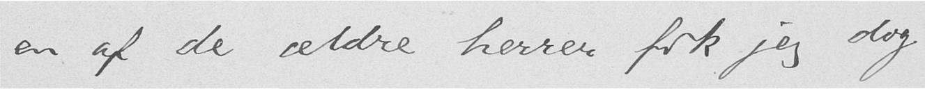en af de ældre herrer fik jeg dog
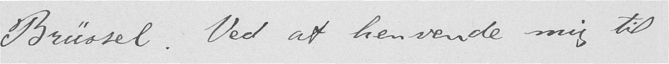Brüssel. Ved at henvende mig til
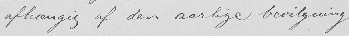afhængig af den aarlige bevilgning.
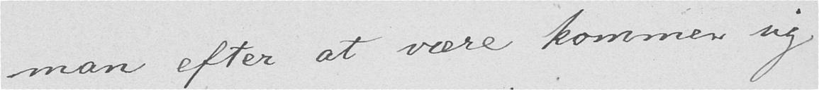man efter at være kommen sig
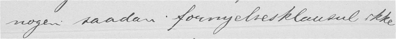nogen saadan fornyelsesklausul ikke
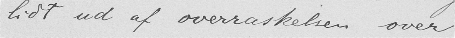lidt ud af overraskelsen over
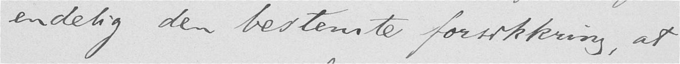endelig den bestemte forsikkring, at
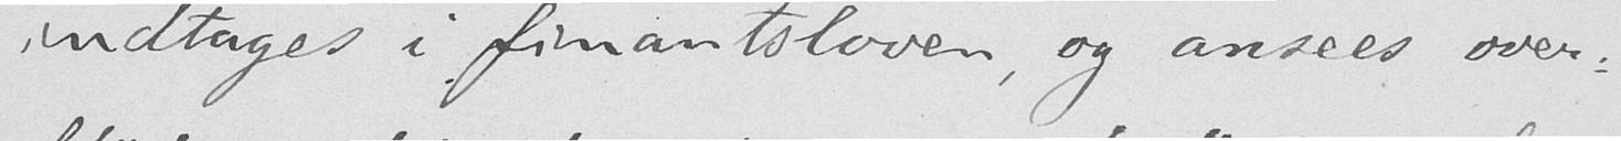indtages i finantsloven, og ansees over-
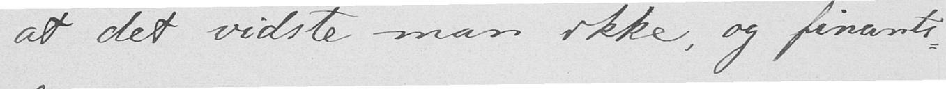at det vidste man ikke, og finants-
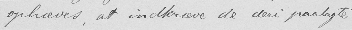ophæves, at indkræve de deri paalagte
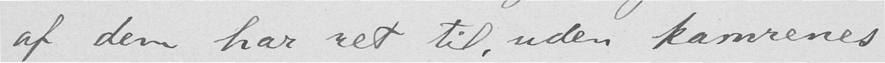af dem har ret til, uden kamrenes
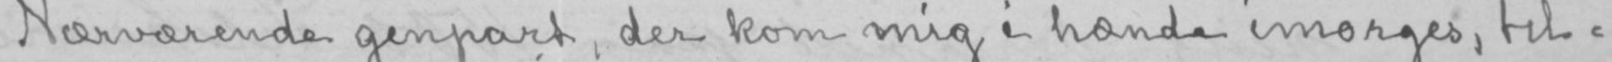Nærværende genpart, der kom mig i hænde imorges, til-
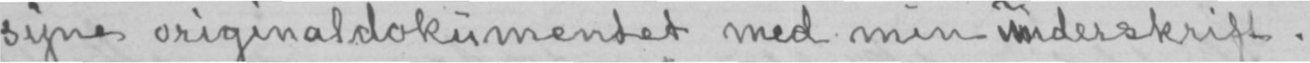syne originaldokumentet med min underskrift.
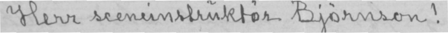Herr sceneinstruktør Bjørnson!
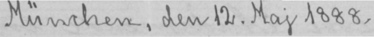München, den 12. Maj 1888.
